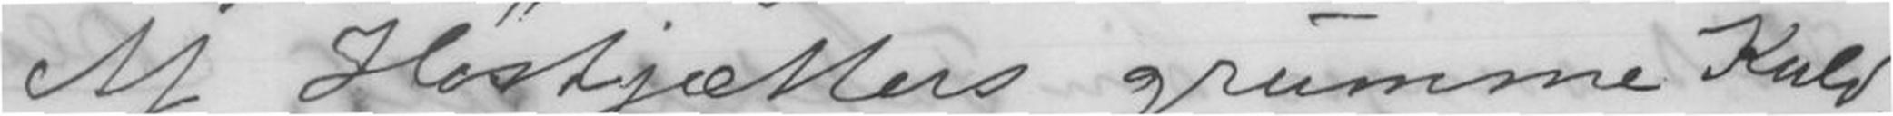Af Høstjætters grumme Kuld.
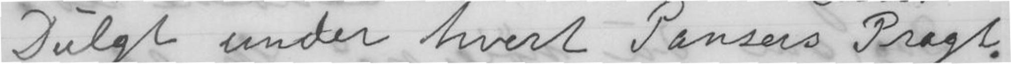Dulgt under hvert Pansers Pragt.
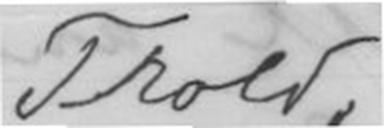Trold,
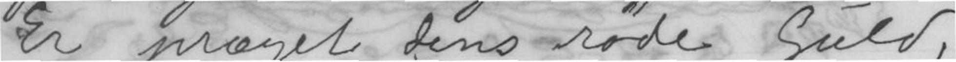Er præget dens røde Guld,
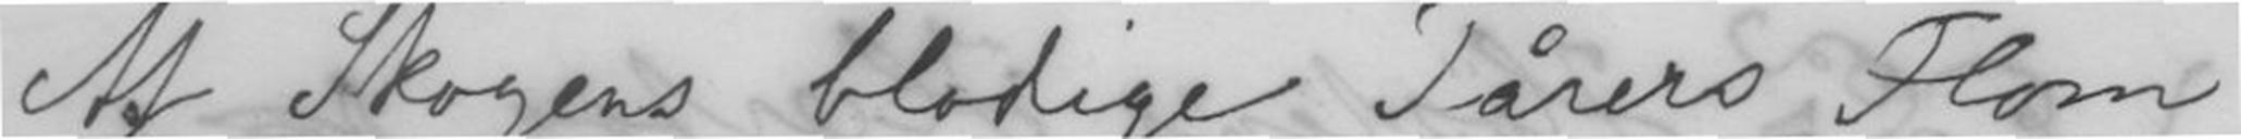Af Skogens blodige Tårers Flom
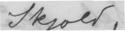Skjold,
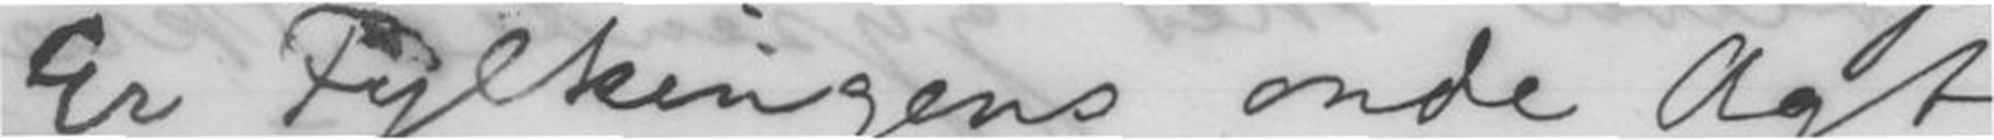Er Fylkingens onde Agt. -
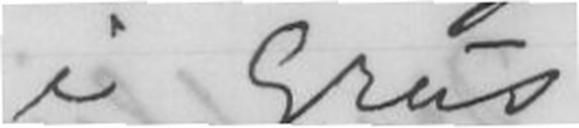i Grus
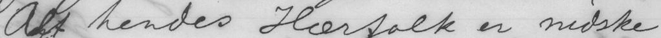Alt hendes Hærfolk er nidske
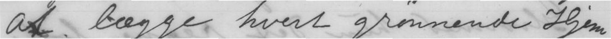At lægge hvert grønnende Hjem
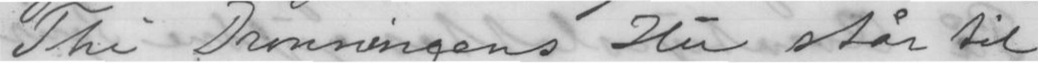Thi Dronningens Hu står til
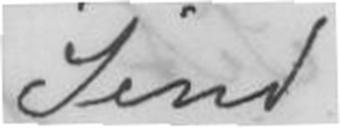Sind
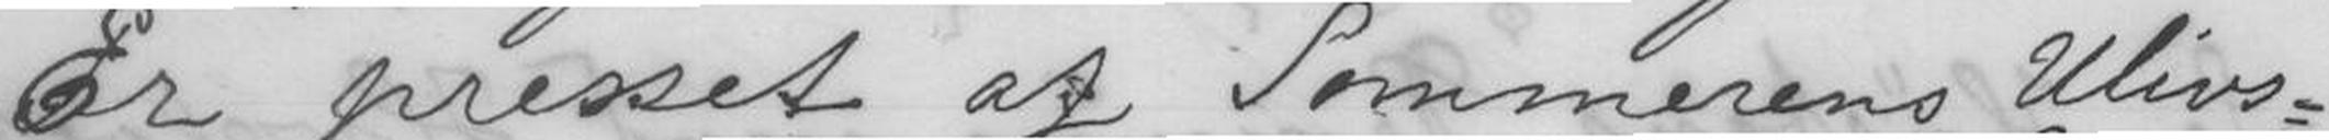Er presset af Sommerens Ulivs-
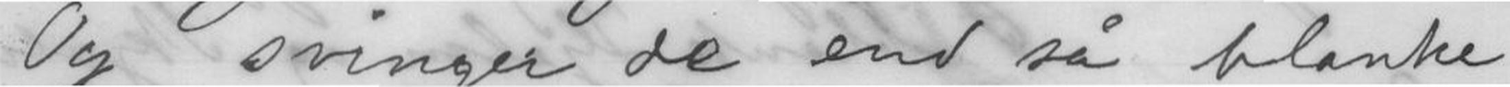Og svinger de end så blanke
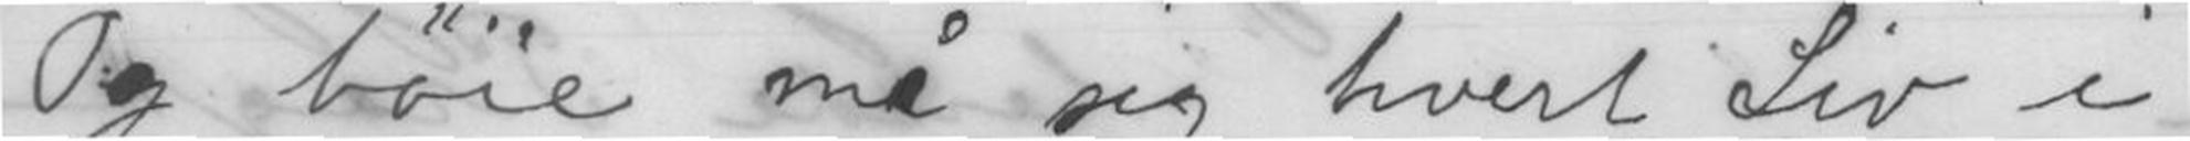Og bøie må sig hvert Liv i
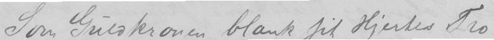Som Guldkronen blank sit Hjertes Tro
